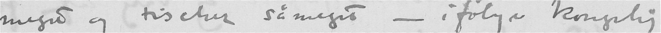meget og Fischer så meget - ifølge kongelig
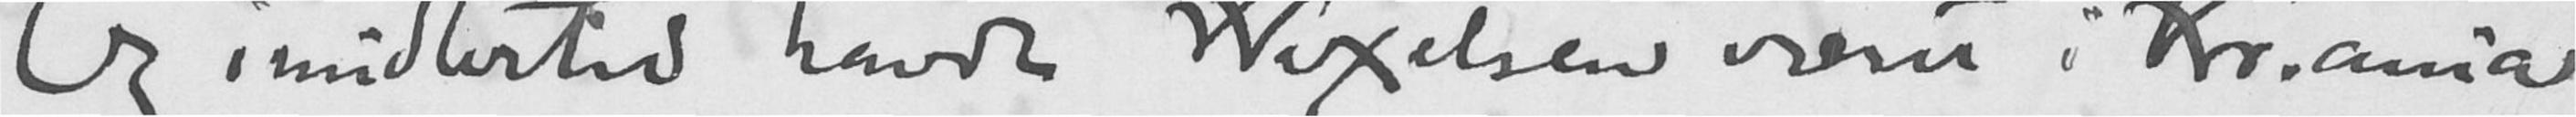Og imidlertid havde Wexelsen været i Kr.ania
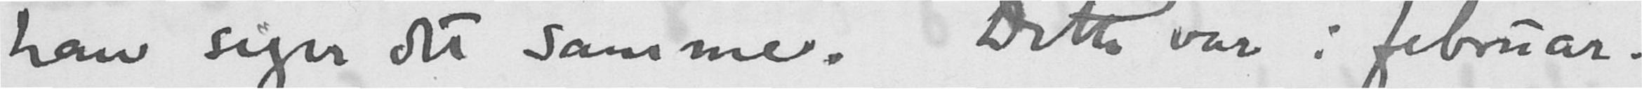han siger det samme. Dette var i februar.
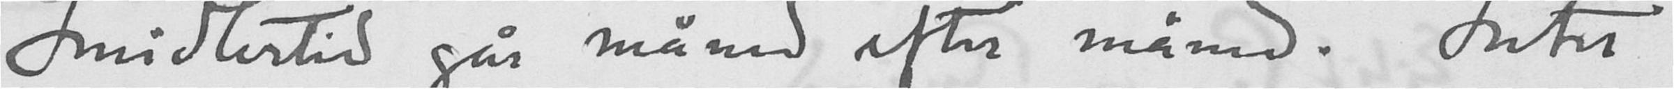Imidlertid går måned efter måned. Intet
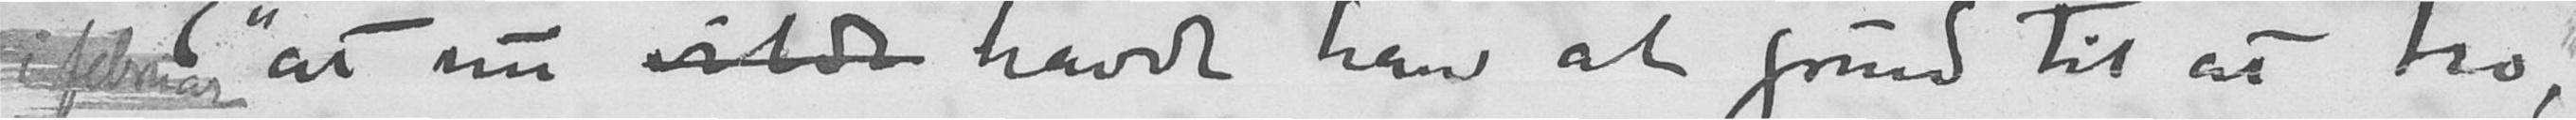i februar "at nu vilde havde han al grund til at tro,
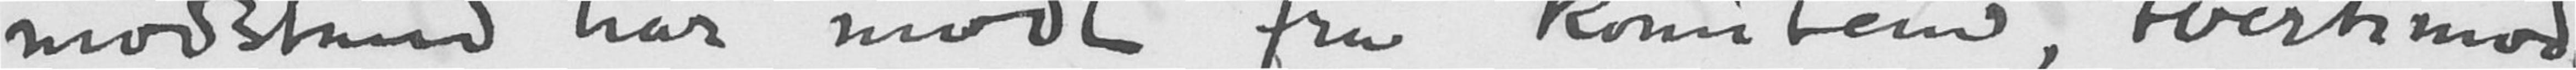modstand har mødt fra komiteen, tvertimod.
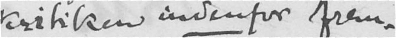kritiken indenfor frem-
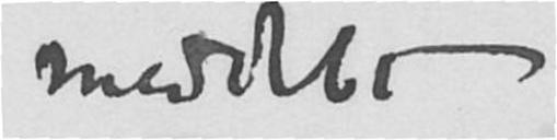meddelt
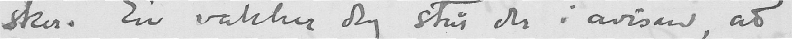sker. En vakker dag står der i avisen, at
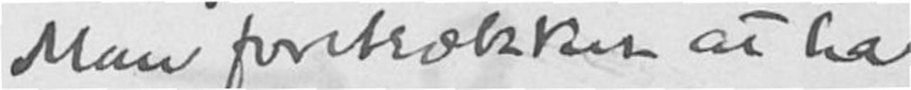Man foretrækker at ha
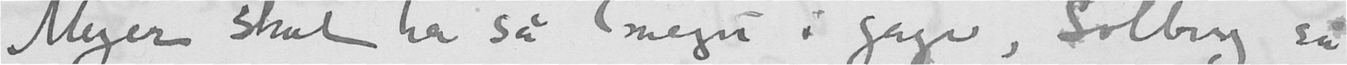Meyer skal ha så meget i gage, Solberg så
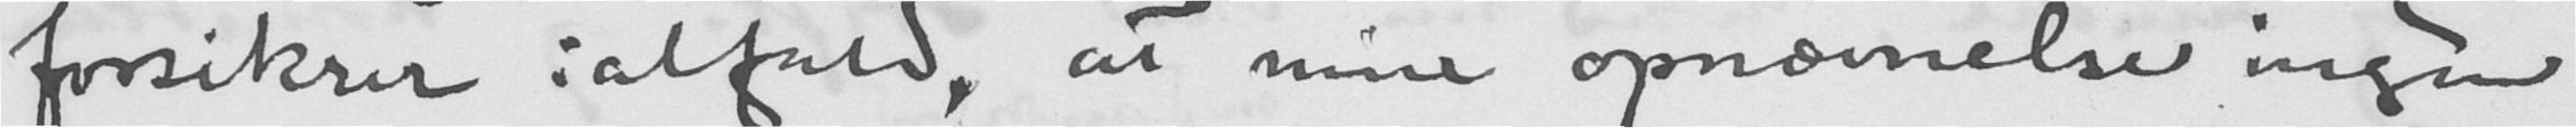forsikrer ialfald, at min opnævnelse ingen
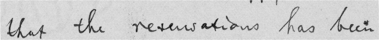that the resensations has been
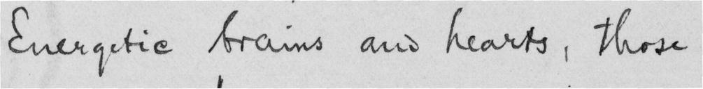Energetic brains and hearts, those
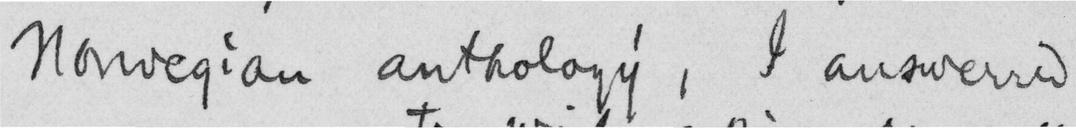Norwegian anthology, I answerred
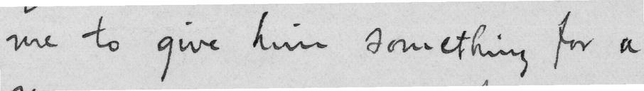me to give him something for a
